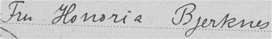Fru Honoria Bjerknes
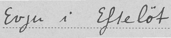Evju i Efteløt
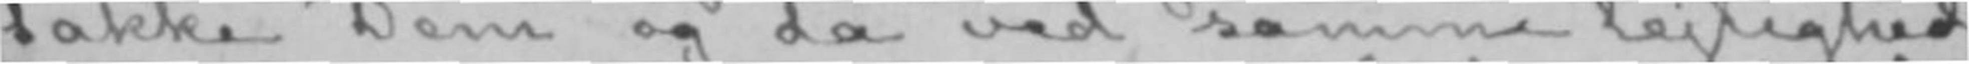takke Dem og da ved samme lejlighed
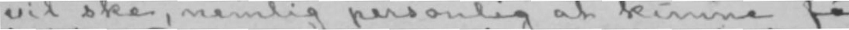vil ske, nemlig personlig at kunne få
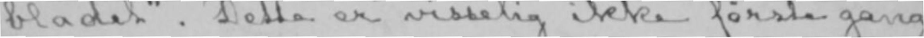bladet». Dette er visselig ikke første gang
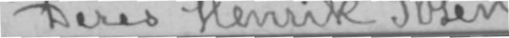Deres Henrik Ibsen.
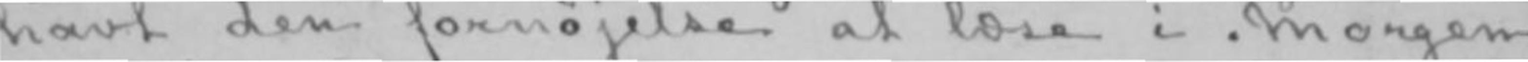havt den fornøjelse at læse i «Morgen-
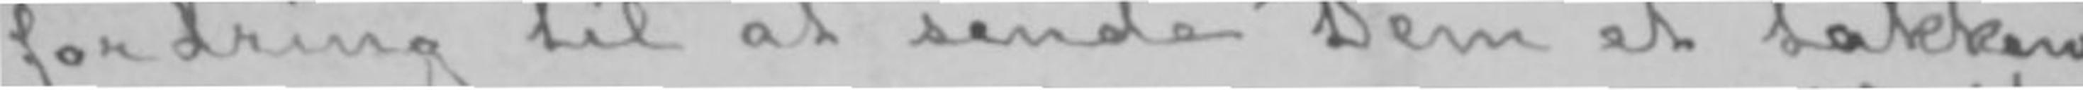fordring til at sende Dem et takkens
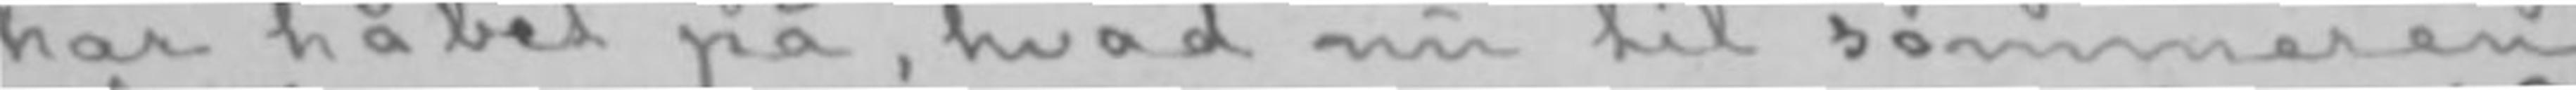har håbet på, hvad nu til sommeren
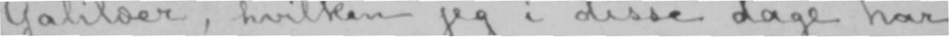Galilæer», hvilken jeg i disse dage har
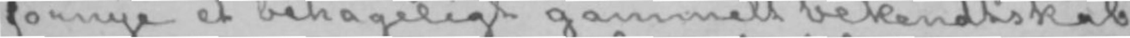fornye et behageligt gammelt bekendtskab.
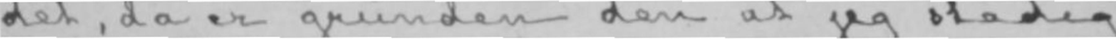det, da er grunden den at jeg stadig
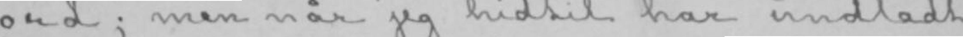ord; men når jeg hidtil har undladt
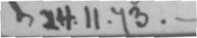d. 24. 11. 73.-
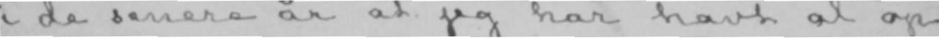i de senere år at jeg har havt al op-
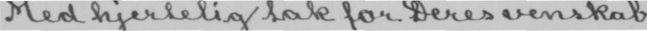Med hjertelig tak for Deres venskab
